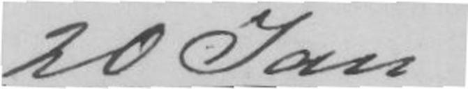20 Jan
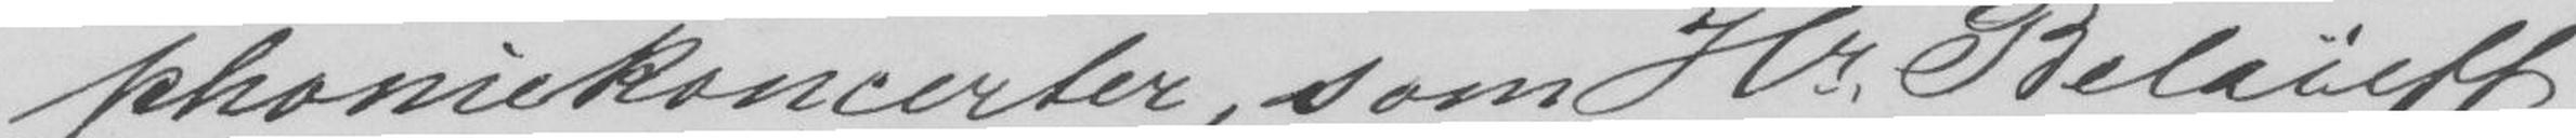phonikoncerter, som Hr. Bela..ff
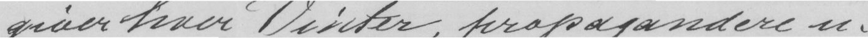giver hver Vinter, propagandere u-
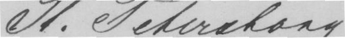St. Petersborg
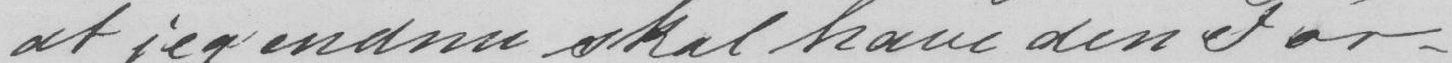at jeg endnu skal have den For-
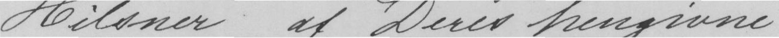Hilsner af Deres hengivne
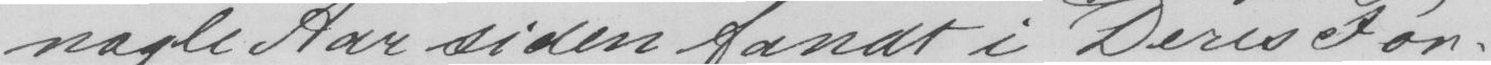nogle Aar siden fandt i Deres For-
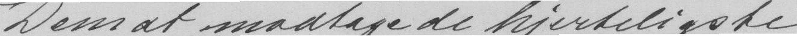Dem at modtage de hjerteligste
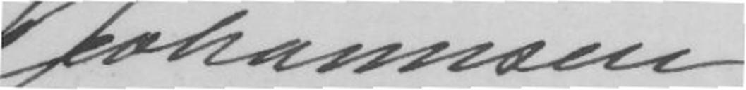JJohannsen
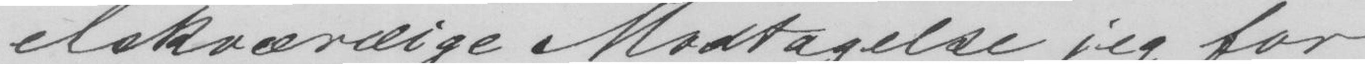elskværdige Modtagelse jeg for
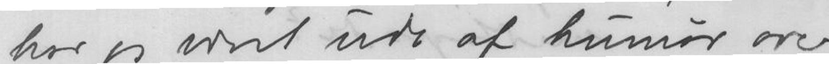har jeg været ude af humør over
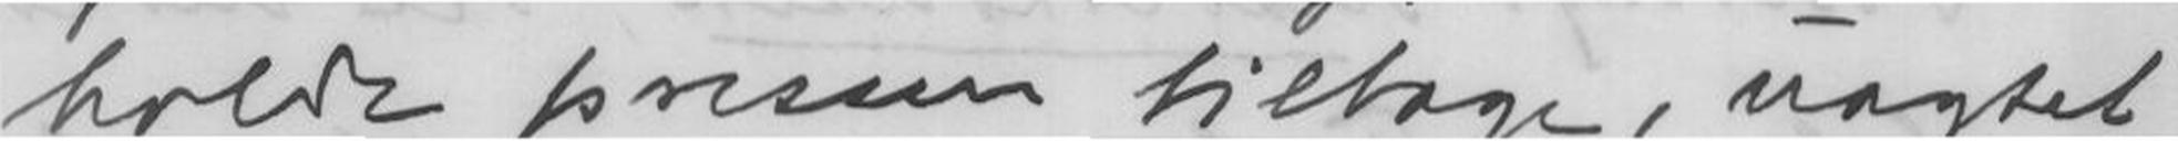holde pressen tilbage, uagtet
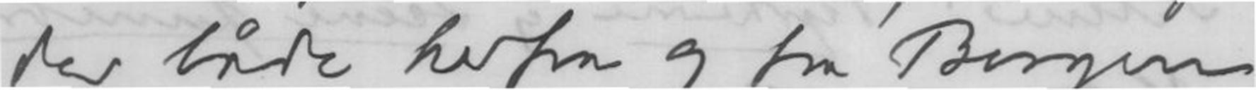der både herfra og fra Bergen
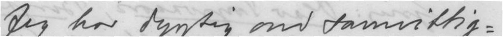Jeg har dygtig med samvittig-
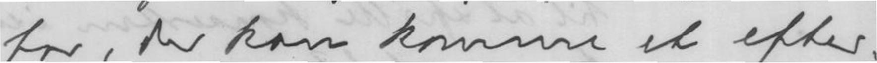for, der kan komme et efter-
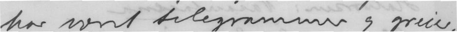har været telegrammer og greier.
